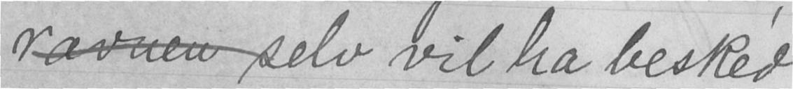ravnen selv vil ha besked
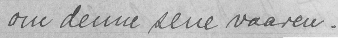om denne sene vaaren.
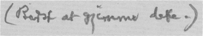(Bedst at gjemme dette.)
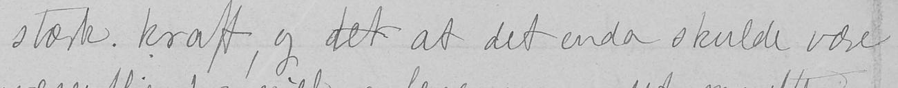stærk kraft, og det at det enda skulde være
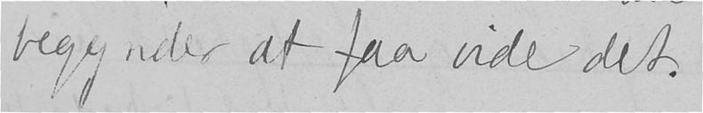begynder at faa vide det.
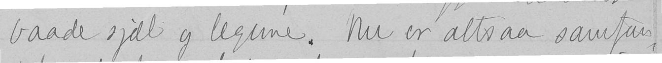baade sjæl og legeme. Nu er altsaa samfun-
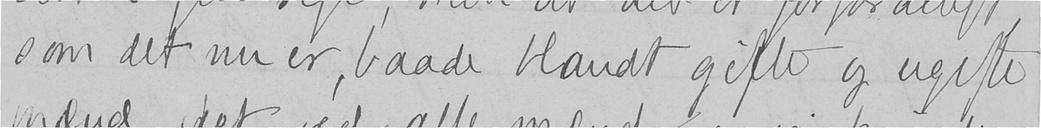som det nu er, baade blandt gifte og ugifte
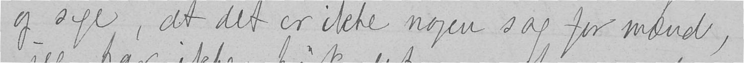og sige, at det er ikke nogen sag for mænd,
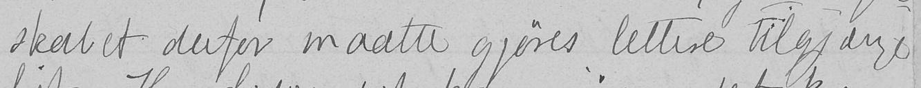skabet derfor maatte gjøres lettere tilgjænge-
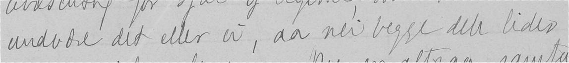undvære det eller ei, aa nei begge dele lider
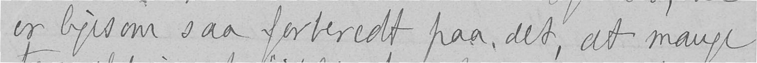er ligesom saa forberedt paa, det, at mange
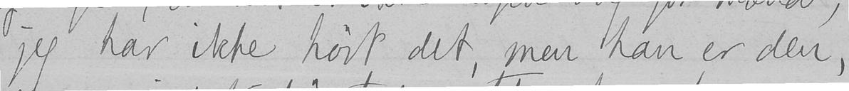jeg har ikke hørt det, men han er den,
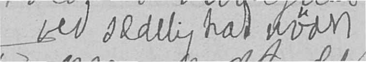ved sædelighet nødv
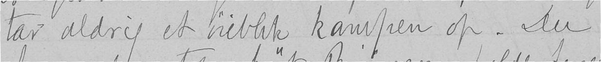tar aldrig et øieblik kampen op. Du
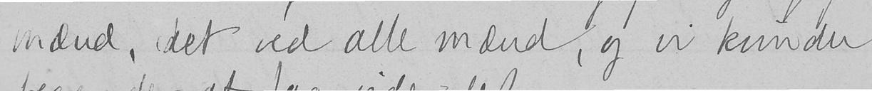mænd, det ved alle mænd, og vi kvinder
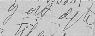og at ægte-
